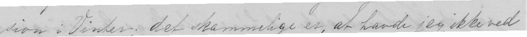sion i Vinter; det skammelige er, at havde jeg ikke ved
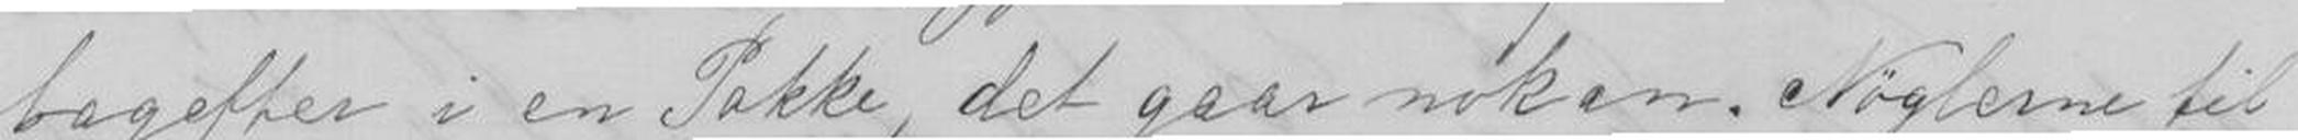bagefter i en Pakke, det gaar noken. Nøglerne til
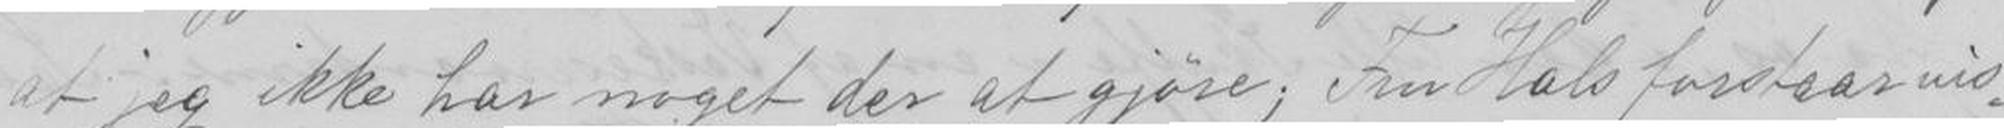at jeg ikke har noget der at gjøre, Fru Hals forstaar vis-
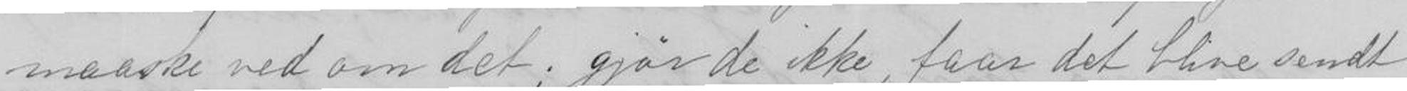maaske ved om det; gjøre de ikke, faar det blive sendt
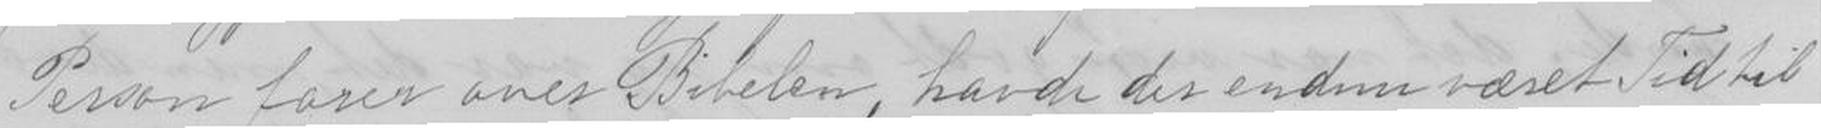Person farer over Bibelen, havde des endun ræset Tid til
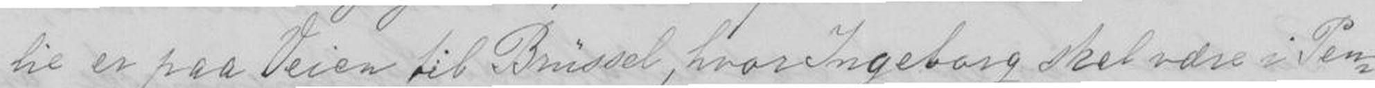lie er paa Veien til Brussel, hvor Ingeborg skal være i Pen-
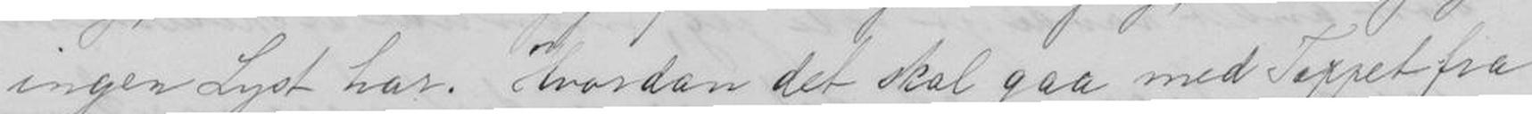ingen lyst har. Hvordan det skal gaa med Teppet fra
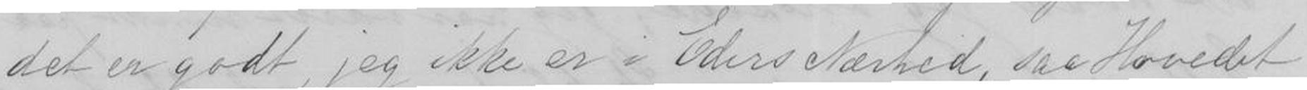det er godt, jeg ikke er i Eders Nærhed, saa Hovedet
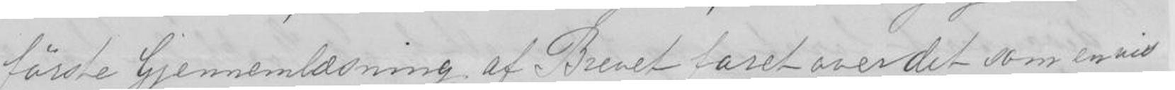første gjennomlæsning af Brevet faret over det som en..
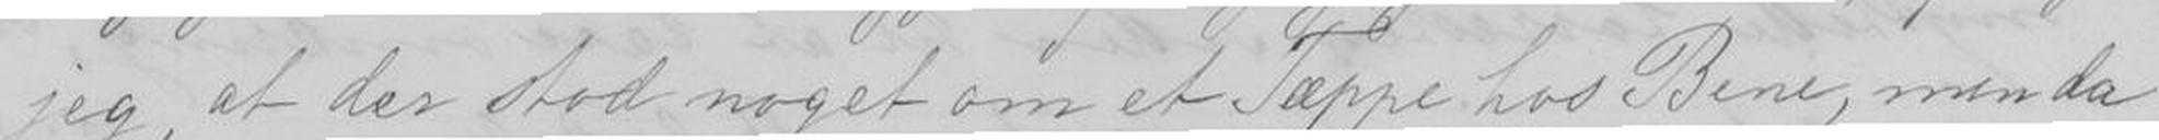jeg, at des stod noget om et Tæppe hos Bene, men da
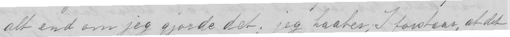alt end om jeg gjorde det; jeg haaber, I forstaar, at det
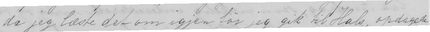da jeg læste det om igjen før jeg gik til hals, opdagede
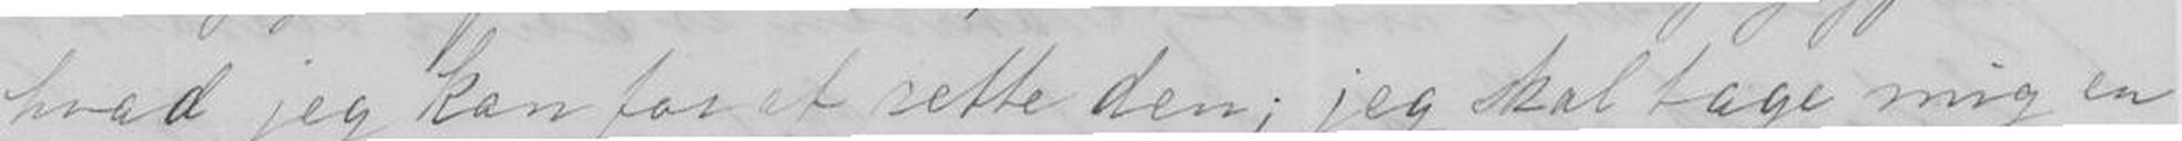hvad jeg kan for at sette den: jeg skal tage mig en
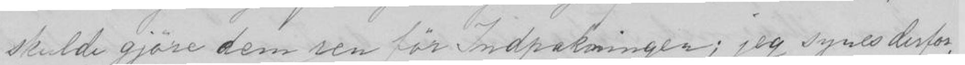skulde gjøre dem ren før Indpaktningen; jeg synes derfor,
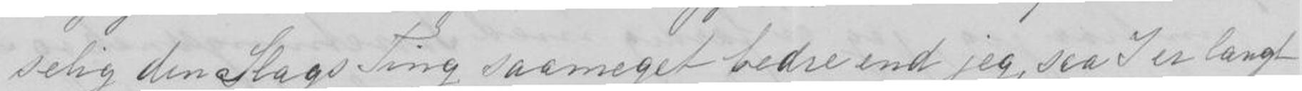selig den Slags ting saa meget bedre end jeg, saa I er langt
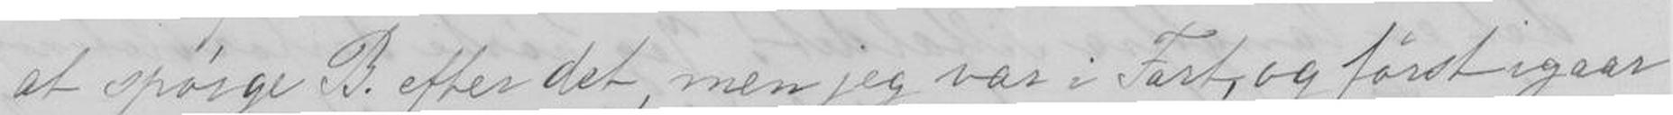at opørge B.efter det, men jeg var i Fart, og først igaar
